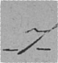- 7 -
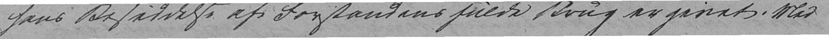hans Besiddelse af Forstandens fulde Brug er givet. Med
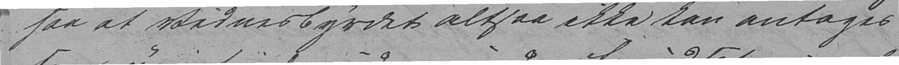saa at Vidnesbyrdet altsaa ikke kan antages
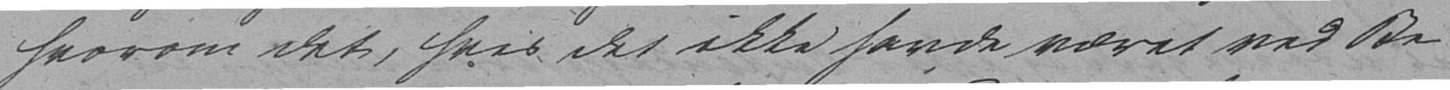hvorom det, hvis det ikke havde været ved Be-
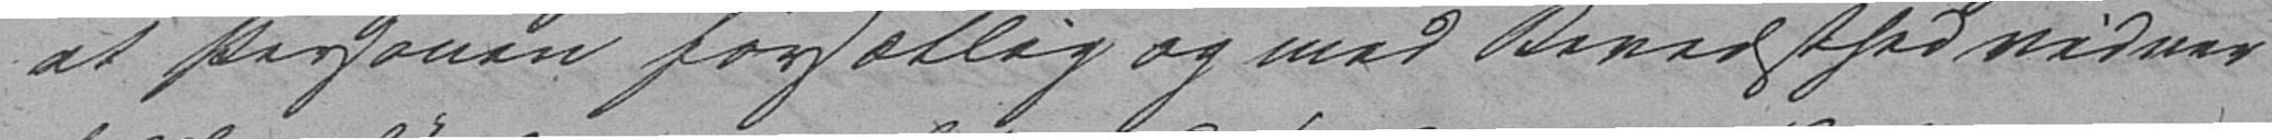at Personen forsætlig og med Bevidsthed vidner
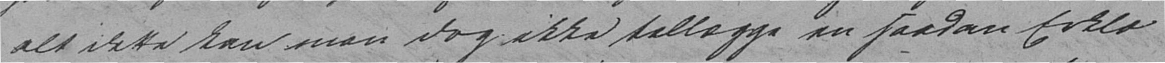alt dette kan man dog ikke tillægge en saadan Erklæ-
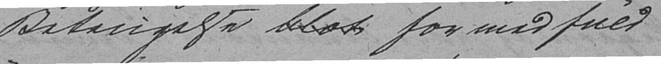Betingelse blot for med fuld
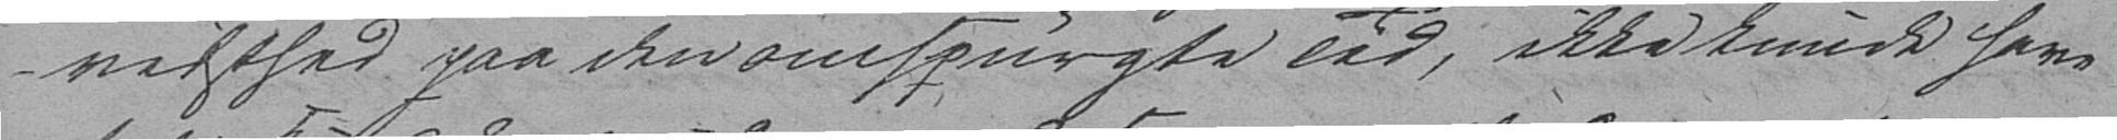vidsthed paa den omspurgte Tid, ikke kunde have
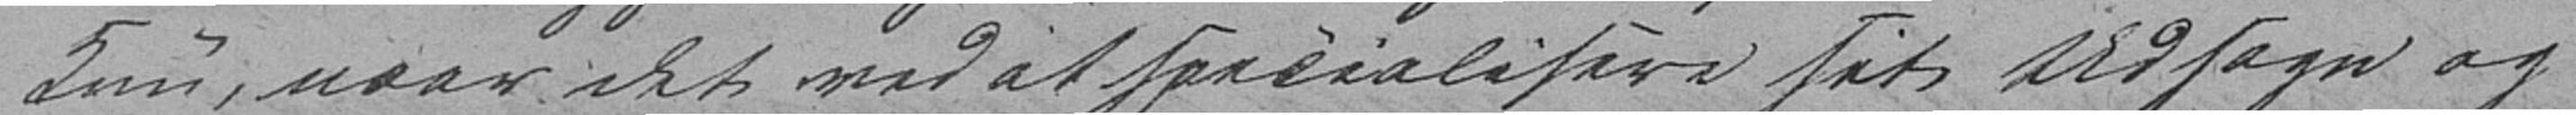Kun, naar det ved at specialisere sit Udsagn og
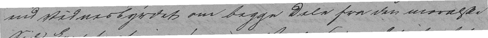end Vidnesbyrdet om begge Dele fra den moralske
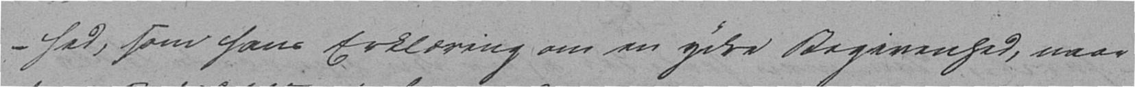hed, som hans Erklæring om en ydre Begivenhed, naar
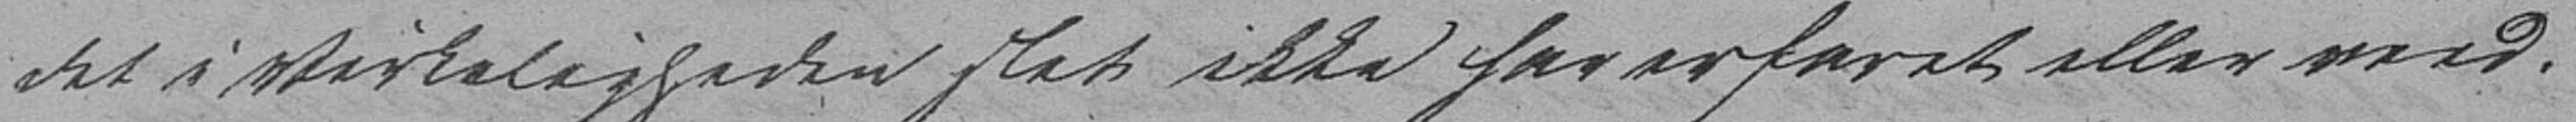det i Virkeligheden slet ikke har erfaret eller veed.
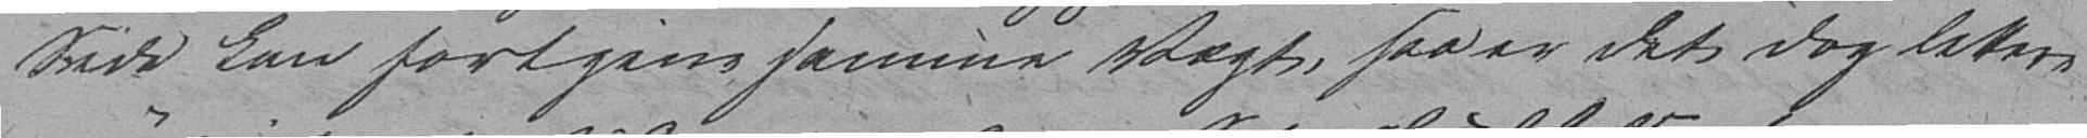Side kan fortjenes samme Vægt, saa er det dog lettere
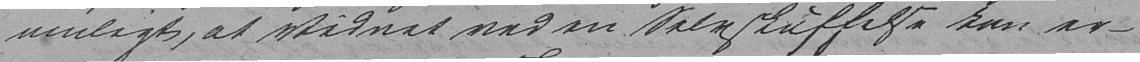muligt, at Vidnet med en Selvskuffelse kan er-
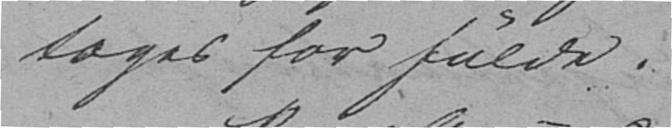tages for fulde.
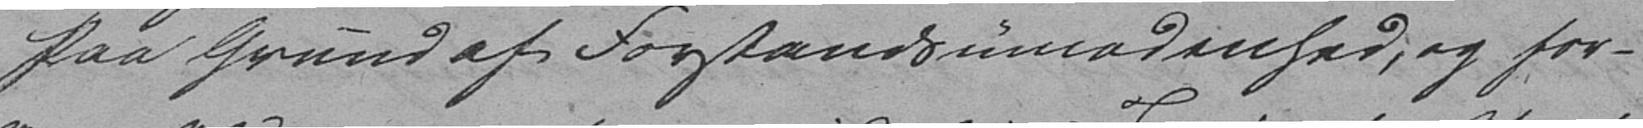Paa Grund af Forstandsumodenhed, og for-
